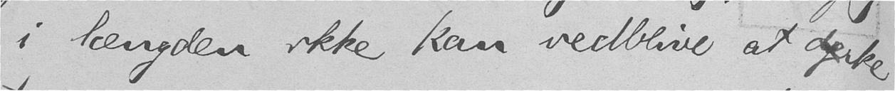i længden ikke kan vedblive at dyrke
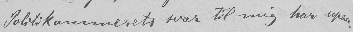Politikammerets svar til mig har upaa-
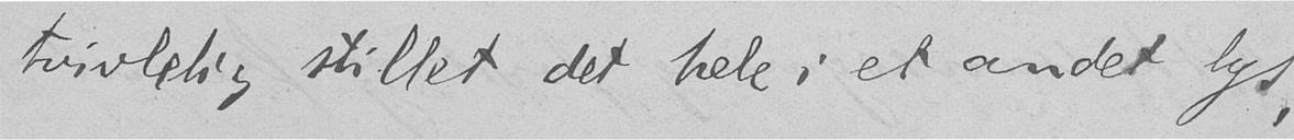tvivlelig stillet det hele i et andet lys,
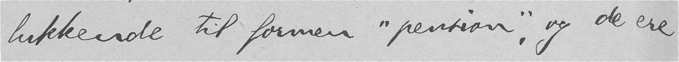lukkende til formen "pension", og de ere
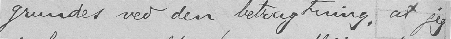grundes ved den betragtning, at jeg
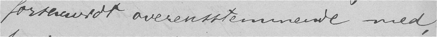forsaavidt overensstemmende med,
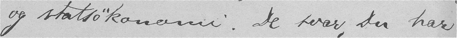og statsøkonomi. De svar, Du har
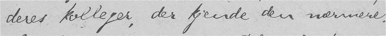deres kolleger, der kjende den nærmere.
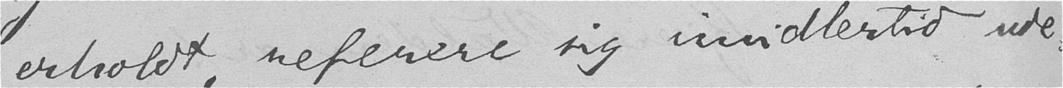erholdt, referere sig imidlertid ude-
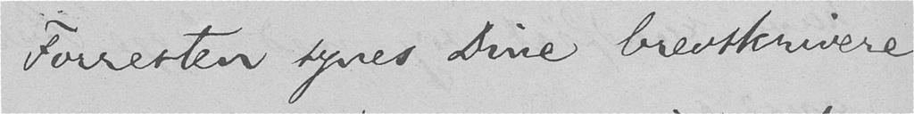Forresten synes Dine brevskrivere
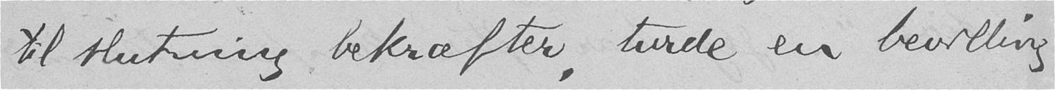til slutning bekræfter, turde en bevilling
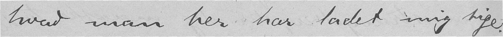hvad man her har ladet mig sige.
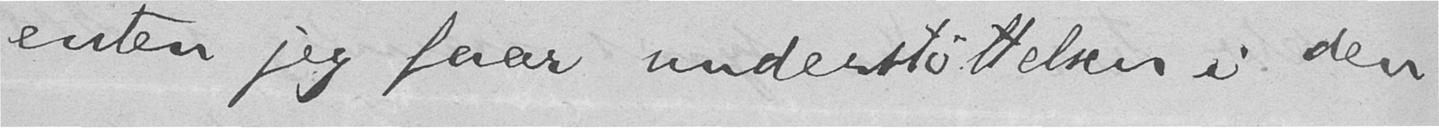enten jeg faar understøttelsen i den
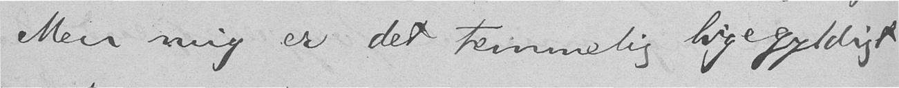Men mig er det temmelig ligegyldigt
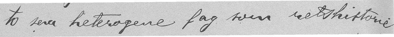to saa heterogene fag som retshistorie
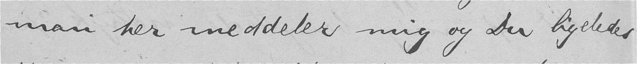man her meddeler mig og Du ligeledes
